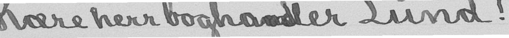Kære herr boghandler Lund!
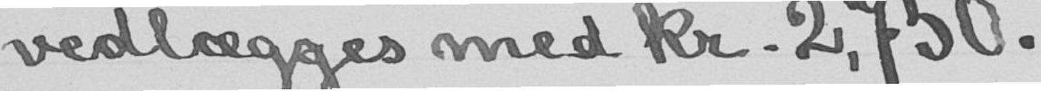vedlægges med kr. 2,750.
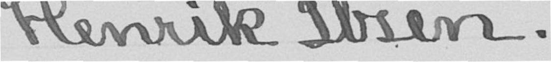Henrik Ibsen.
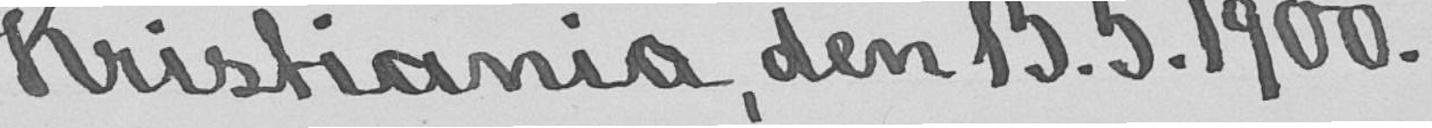Kristiania, den 15.5.1900.
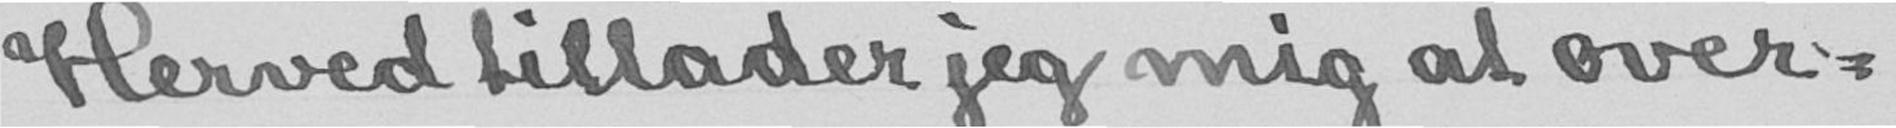Herved tillader jeg mig at over-
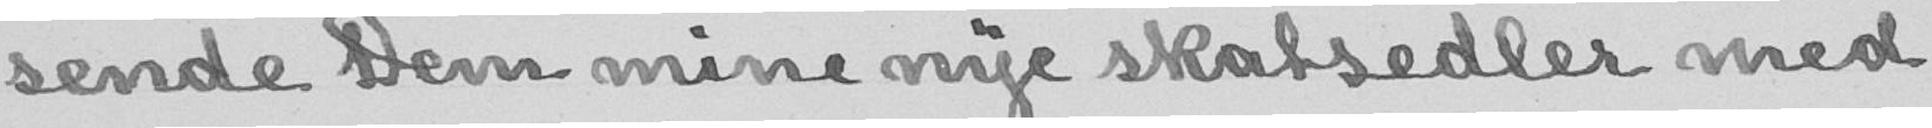sende Dem mine nye skatsedler med
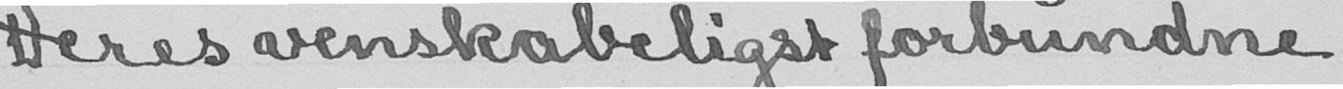Deres venskabeligst forbundne
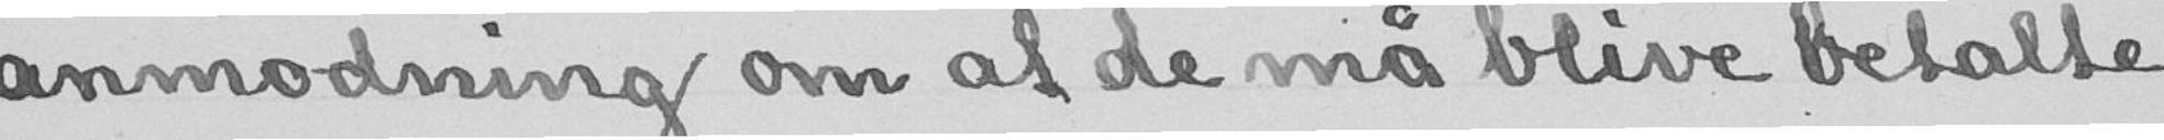anmodning om at de må blive betalte
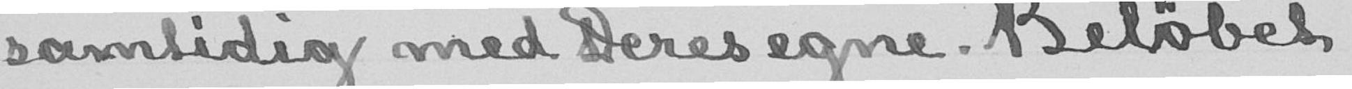samtidig med Deres egne. Beløbet
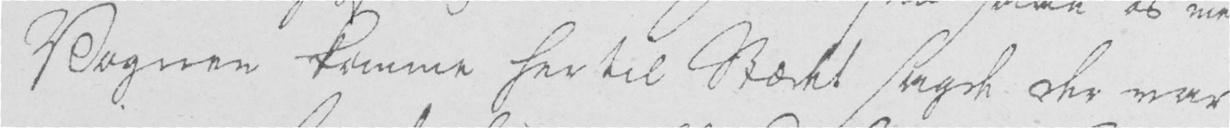Vognen komme her til Stædet sagde der var
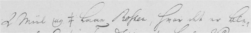2 Miil og 1/4 laae Rosten, hvor det er ble-
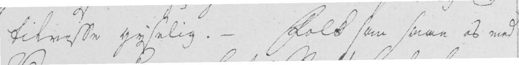tilvisse gyselig. - Folk som saae os med
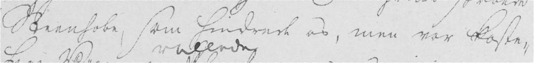Steenhobe, som hindrede os, men vor koste-
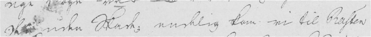dette uden Skade; endelig kom vi til Rosten
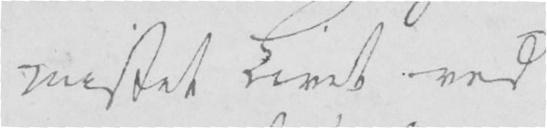mistet Livet ved
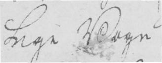lige Vogn
